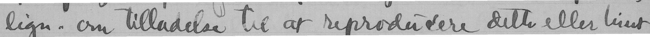lign. om tilladelse til at reproducere dette eller hint
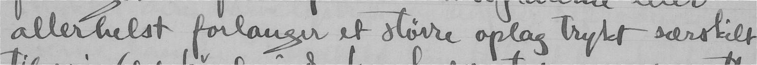allerhelst forlanger et større oplag trykt særskilt
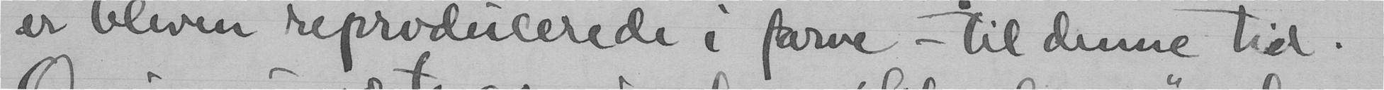er bleven reproducerede i farve - til denne tid.
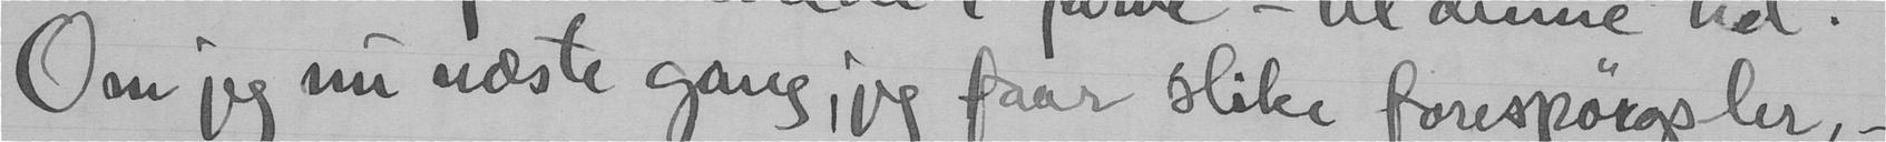Om jeg nu næste gang, jeg faar slike forespørgsler,-
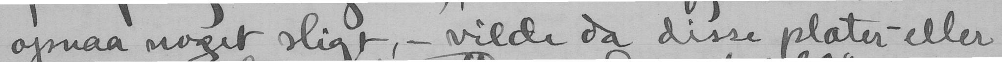opnaa noget sligt, - vilde da disse plater - eller
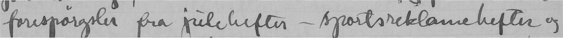forespørgsler fra julehefter - sportsreklamehefter og
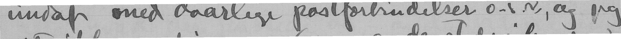undaf med daarlige postforbindelser o.s.v, og jeg
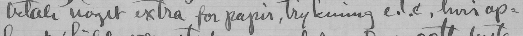betale noget extra for papir, trykning e.t.c, hvir op-
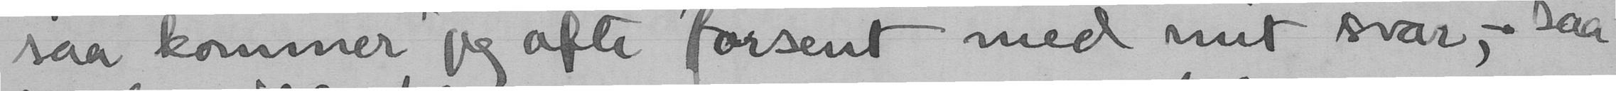saa kommer jeg ofte forsent med mit svar, - saa
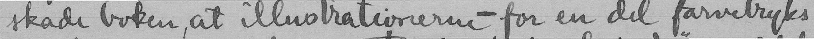skade boken, at illustrationerne - for en del farvetryks
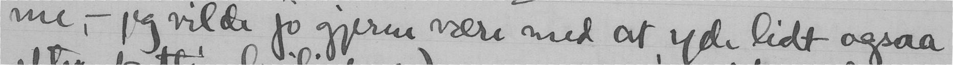me, - jeg vilde jo gjerne være med at yde lidt ogsaa
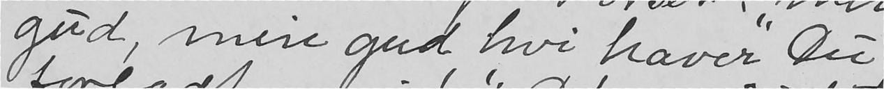gud, min gud hvi haver Du
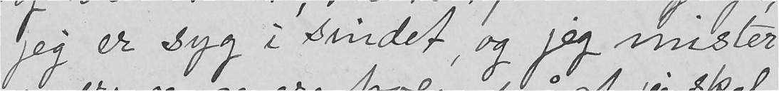jeg er syg i Sindet, og jeg mister
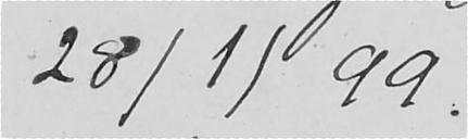28/1/ 99.
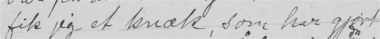fik jeg et knæk, som har gjort
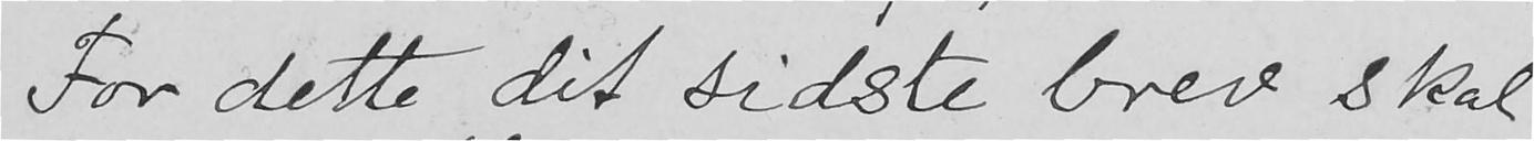For dette dit sidste brev skal
